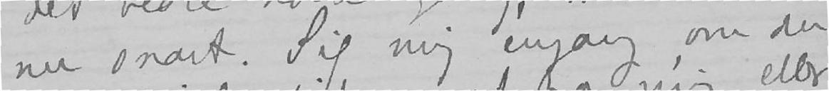nu snart. Sig mig engang, om du
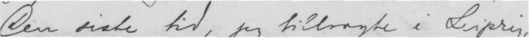Den siste tid, jeg tilbragte i Leipzig,
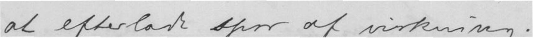at eftelade spor af virkning.
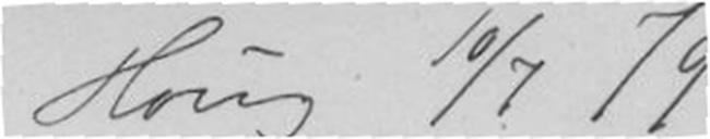Houg 10/7 79
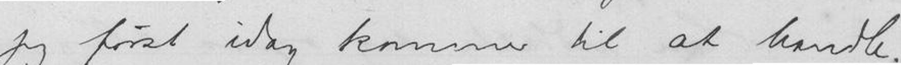jeg først idag kommer til at handle.
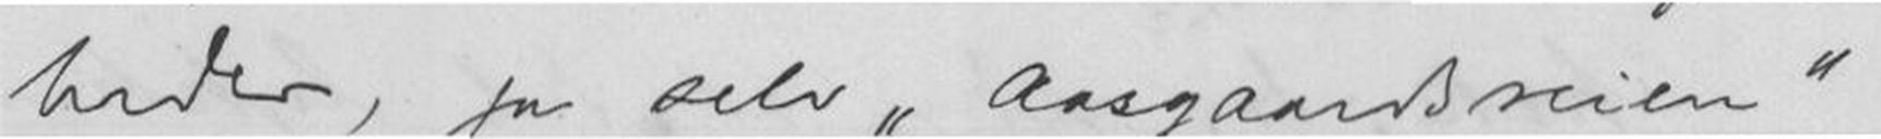heder, ja selv "Aasgaardsreien"
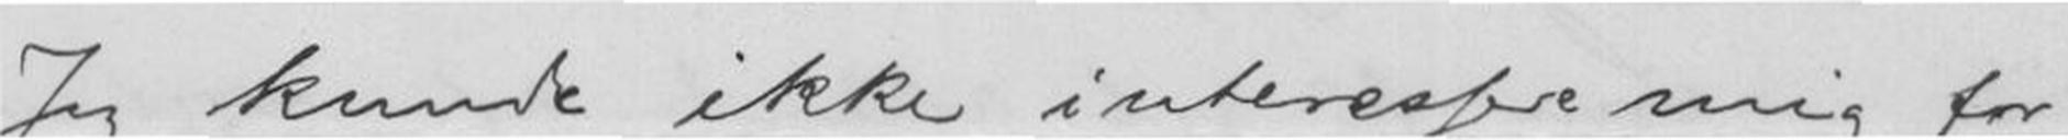Jeg kunde ikke interessere mig for
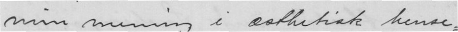min mening i æsthetisk hense
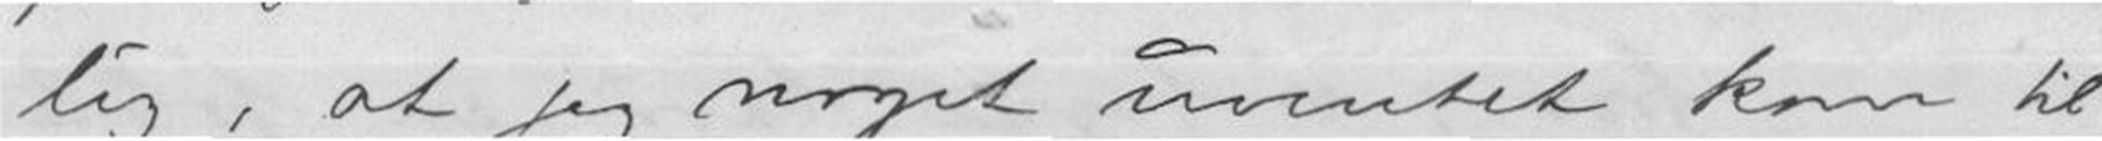lig, at jeg noget uventet kom til
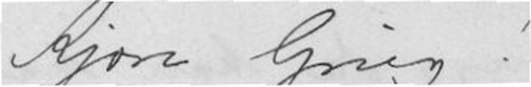Kjære Grieg!
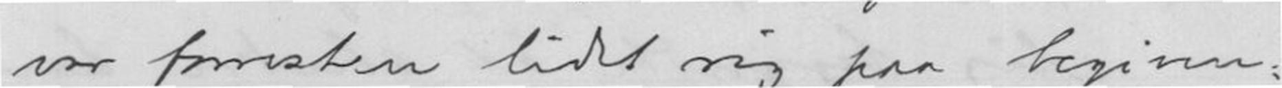var forresten lidt rig paa begiven-
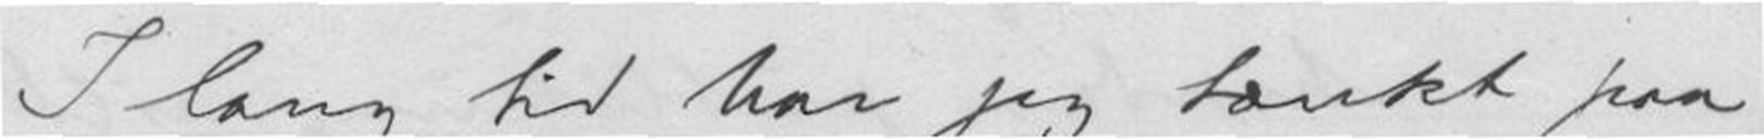I lang tid har jeg tænkt paa
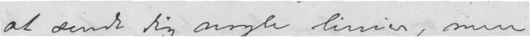at sende dig nogle linier, men
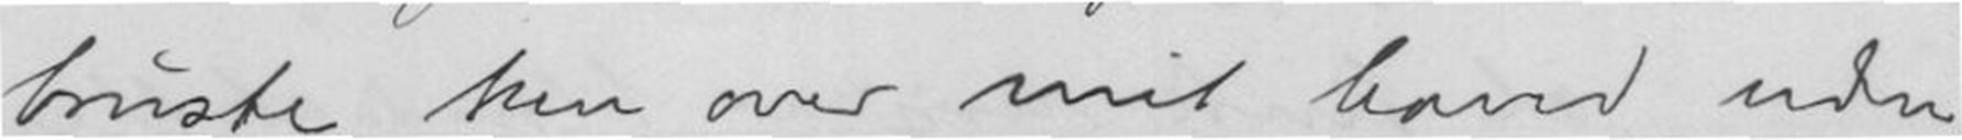bruste hen over mit hoved uden
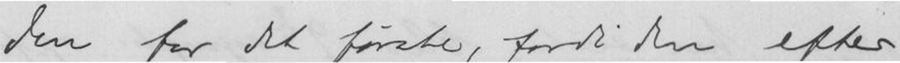den for det første, fordi den efter
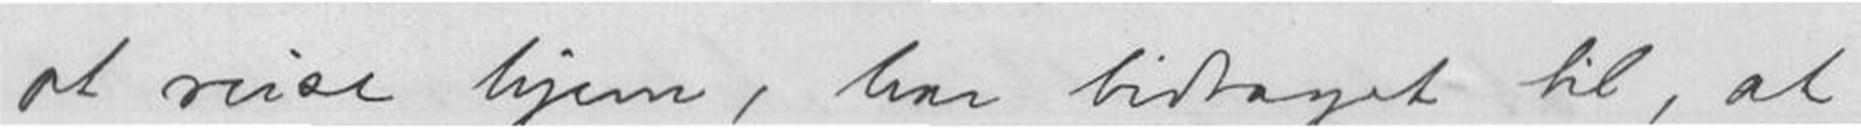at reise hjem, har bidraget til, at
